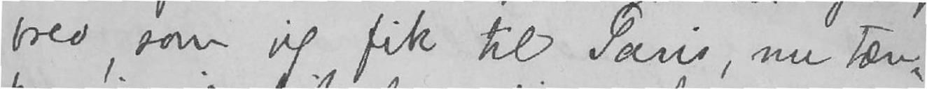brev, som jeg fik til Paris, nu tæn-
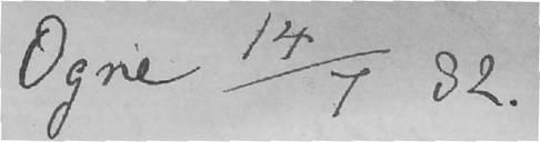Ogne 14/7 82
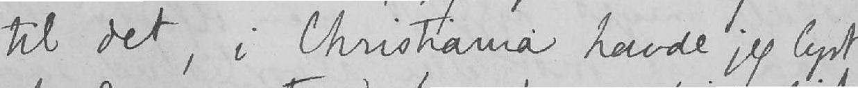til det, i Christiania havde jeg lyst
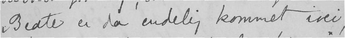Beate er da endelig kommet ivei,
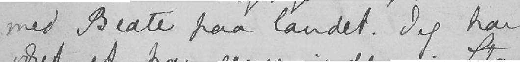med Beate paa landet. Jeg har
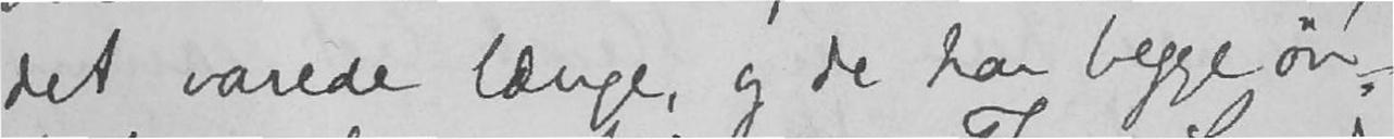det varede længe, og de har begge øn-
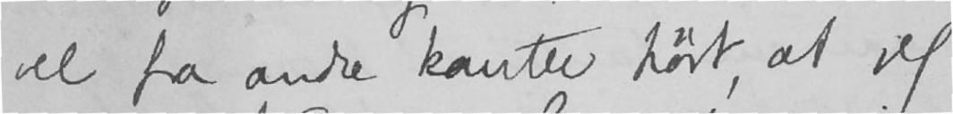vel fra andre kanter hørt, at jeg
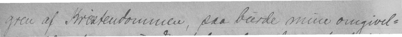gren af Kristendommen, saa burde mine omgivel-
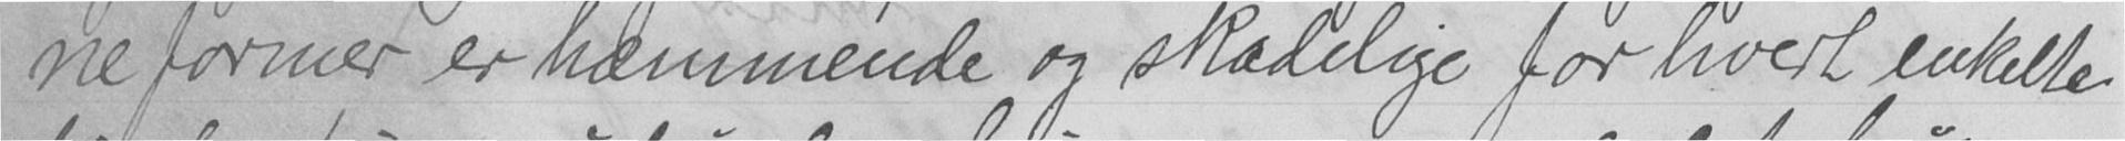ne former er hæmmende og skadelige for hvert enkelte
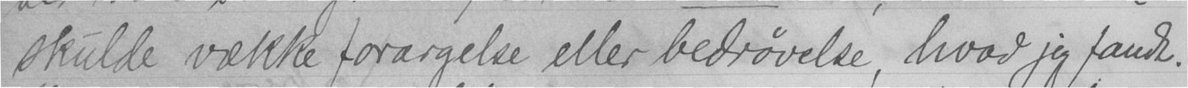skulde vække forargelse eller bedrøvelse, hvad jeg fandt.
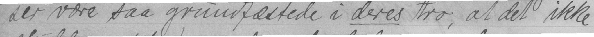ser være saa grundfæstede i deres tro, at det ikke
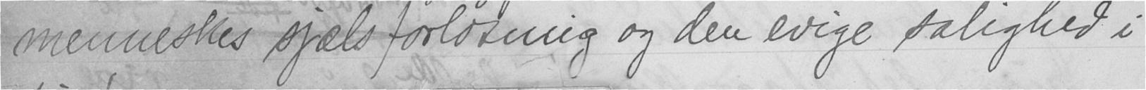menneskes sjæls forløsning og den evige salighed i
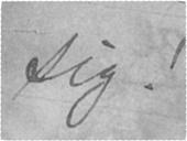sig!
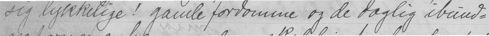sig lykkelige! gamle fordomme og de daglig ibund-
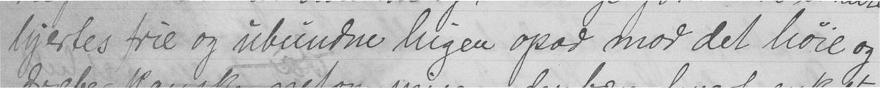hjertes frie og ubundne higen opad mod det høie og
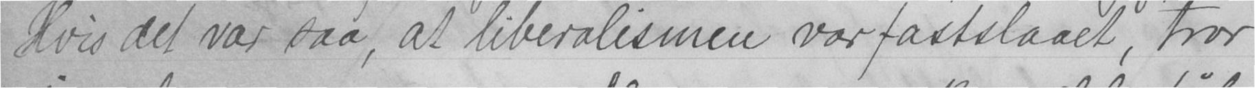Hvis det var saa, at liberalisimen var fastslaaet, tror
